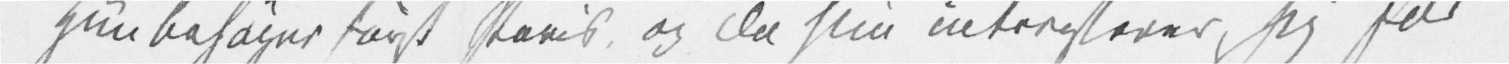Hun besøger først Paris, og da hun interesserer sig for
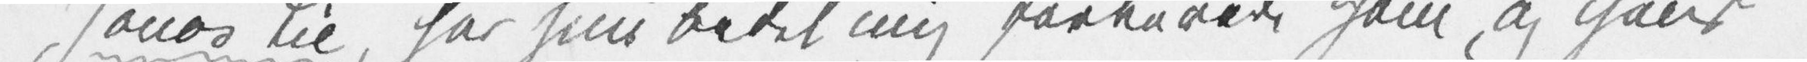Jonas Lie, har hun bedet mig forberede ham og hans
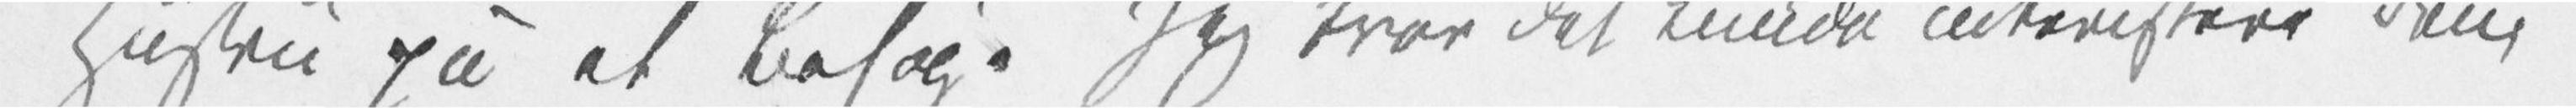Hustru på et Besøg. Jeg tror det kunde interessere Dem
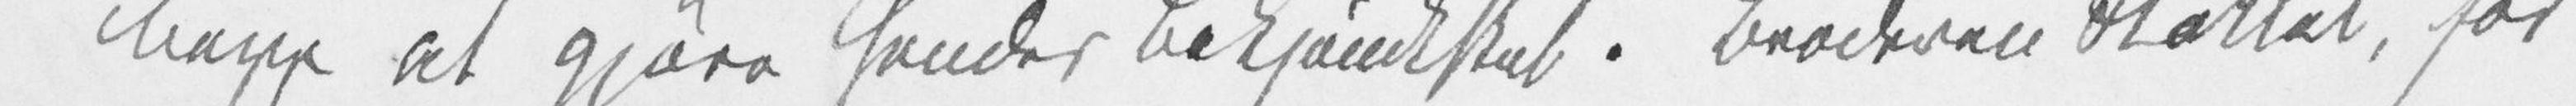begge at gjøre hendes Bekjendtskab. Broderen Stakkel, for
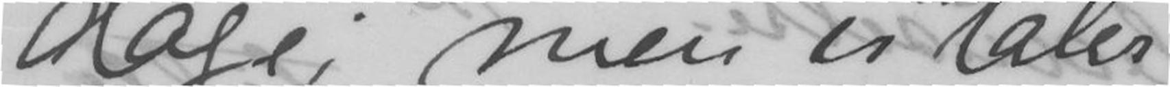dage; men vi taler
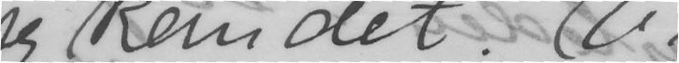jeg kan det. Vi
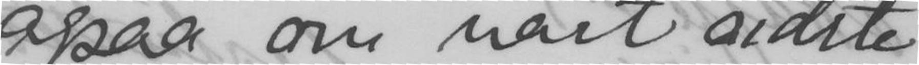ogsaa om vort sidste
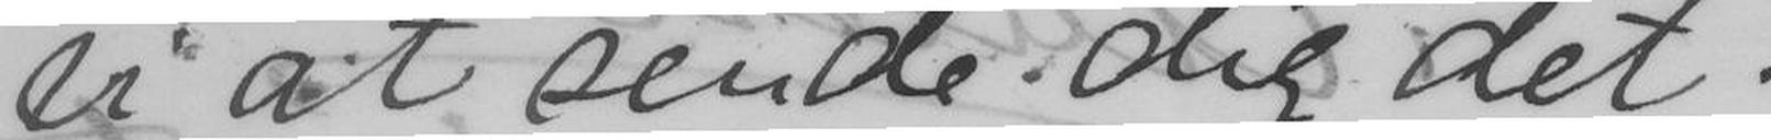vi at sende dig det.
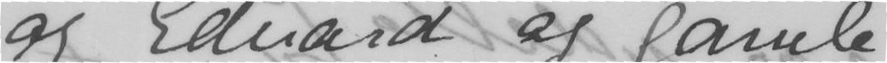og Edvard og gamle
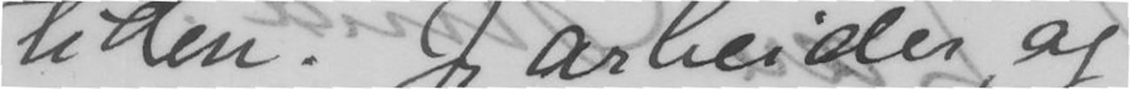tiden. Jeg arbeider, og
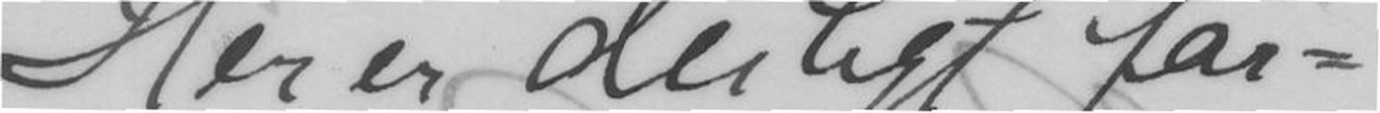Her er deiligt for=
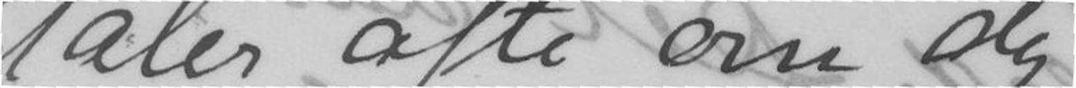taler ofte om Dig
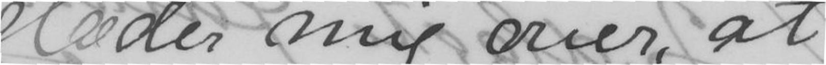glæder mig over, at
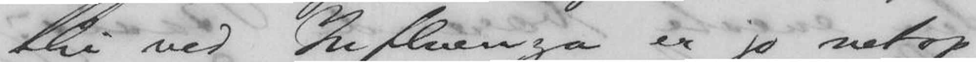thi ved Influenza er jo netop
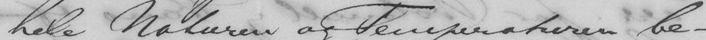hele Naturen og Temperaturen be-
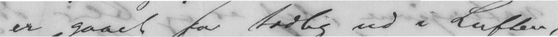er gaaet for tidlig ud i Luften,
